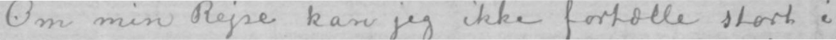Om min Rejse kan jeg ikke fortælle stort i
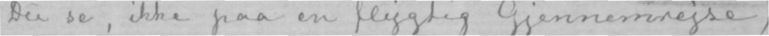Du se, ikke paa en flygtig Gjennemrejse,
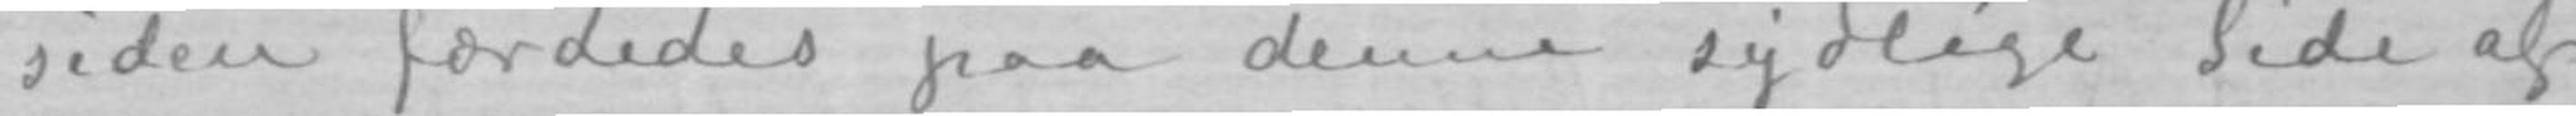siden færdedes paa denne sydlige Side af
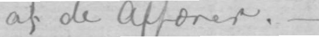af de Affærer.-
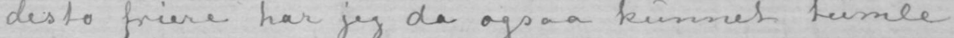desto friere har jeg da ogsaa kunnet tumle
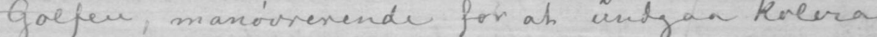Golfen, manøvrerende for at undgaa Kolera
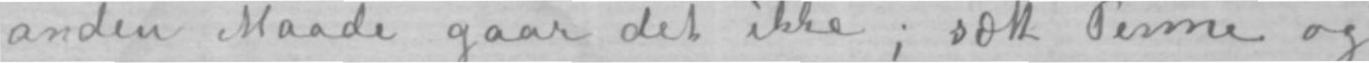anden Maade gaar det ikke; sætt Penne og
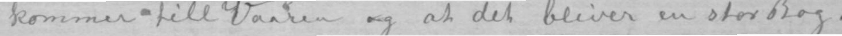kommer till Vaaren og at det bliver en stor Bog.
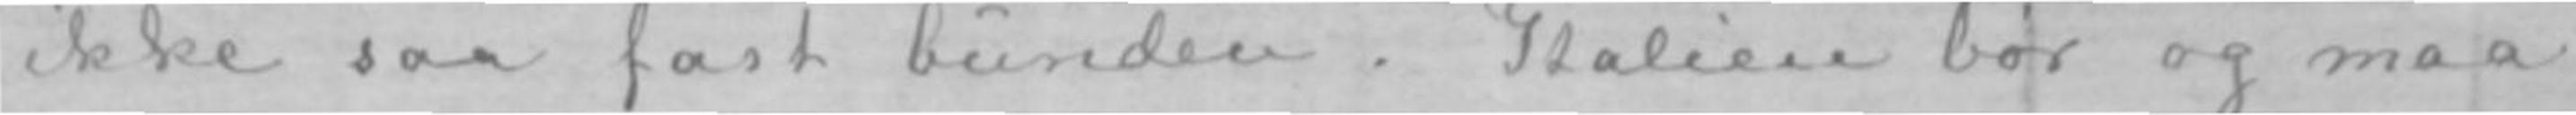ikke saa fast bunden. Italien bør og maa
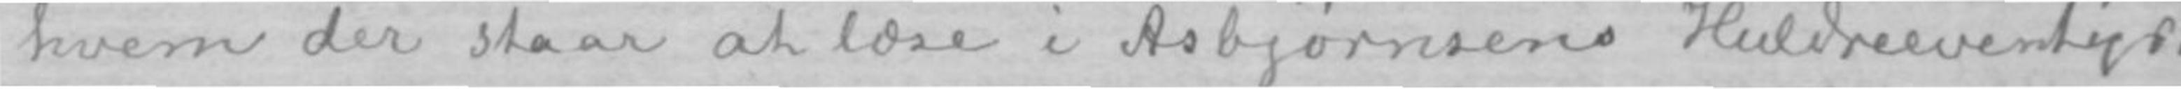hvem der staar at læse i Asbjørnsens Huldreeventyr.
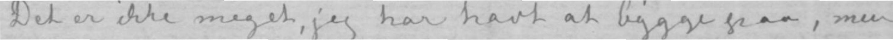Det er ikke meget, jeg har havt at bygge paa, men
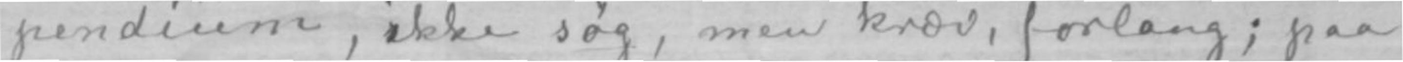pendium, ikke søg, men kræv, forlang; paa
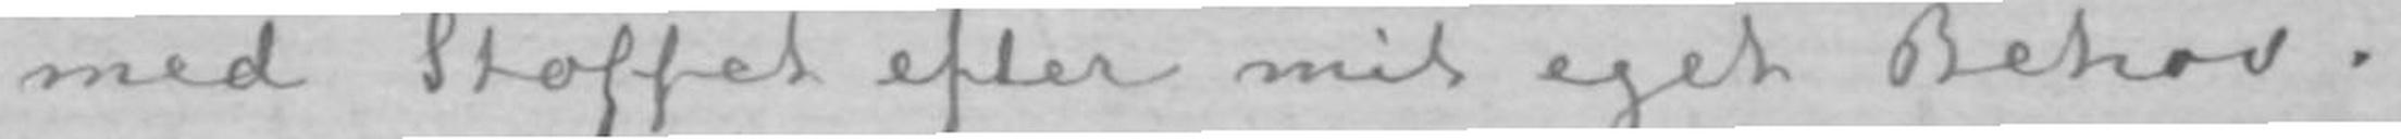med Stoffet efter mit eget Behov.
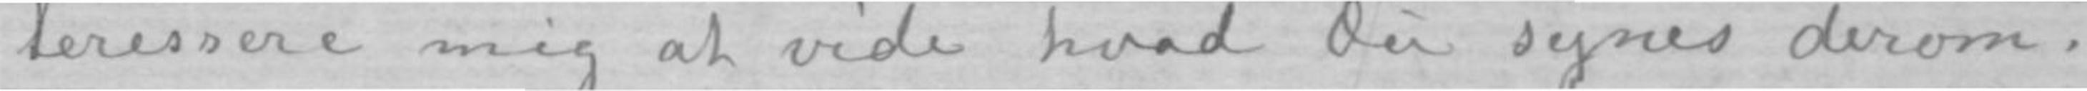teressere mig at vide hvad Du synes derom.
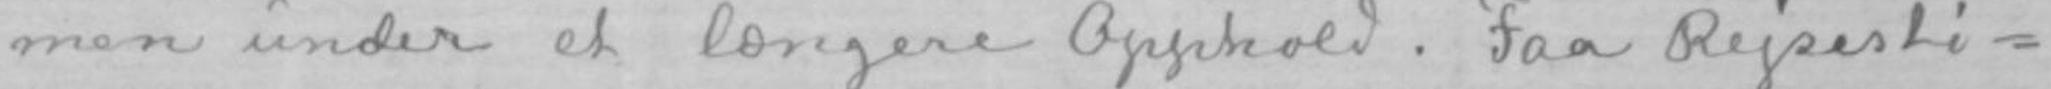men under et længere Opphold. Faa Rejsesti-
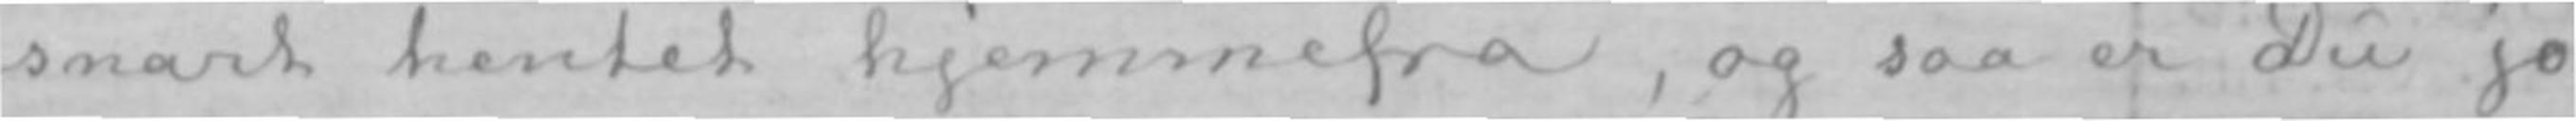snart hentet hjemmefra, og saa er Du jo
